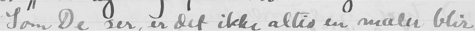Som De ser, er det ikke altid en maler blir
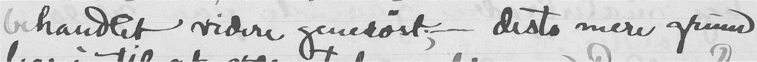behandlet videre generøst; - desto mere grund
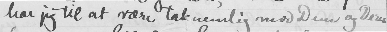har jeg til at være taknemlig mod Dem og Deres
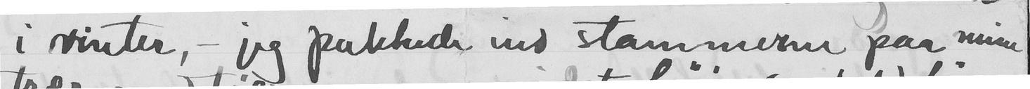i vinter, - jeg pakkede ind stammerne paa mine
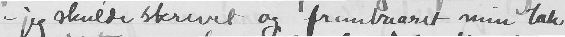- jeg skulde skrevet og frembaaret min tak
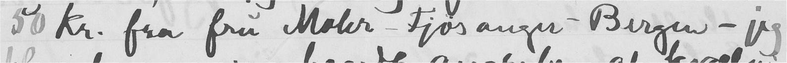50 kr. fra fru Mohr - Fjøsanger - Bergen - jeg
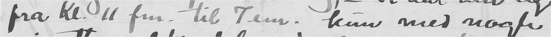fra Kl. 11 fm. til 7 em. kun med nogle
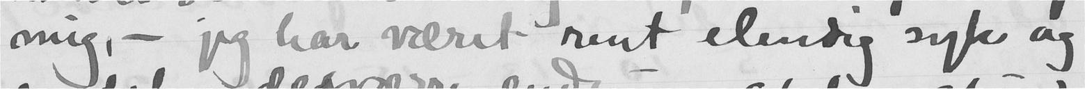mig, - jeg har været rent elendig syk og
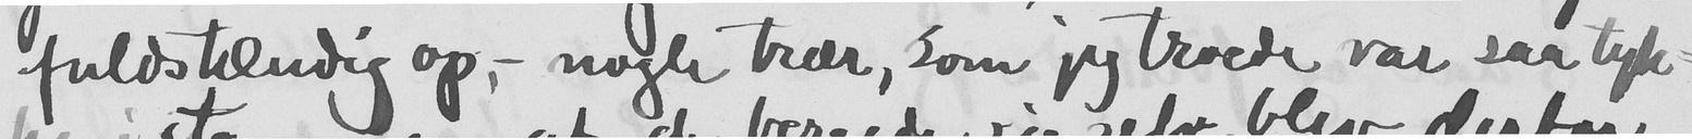fuldstændig op, - nogle trær, som jeg troede var saa tyk-
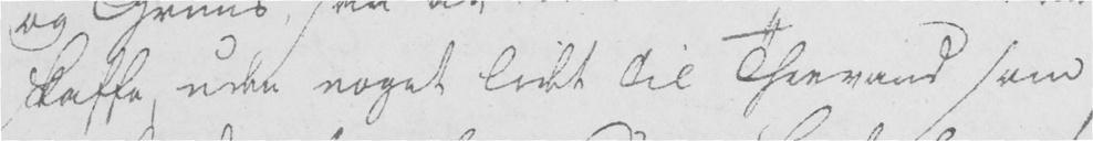skaffe uden noget lidet til Theevand som
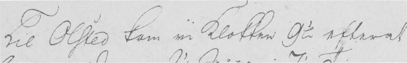Til Olsted kom vi Klokken 9 1/2 efterat
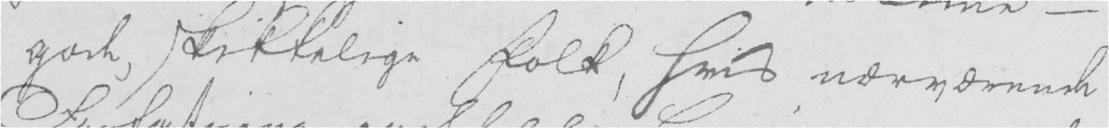gode, skikkelige Folk, hvis nærværende
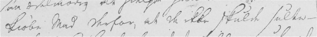kiøbe Mad derfor, at de ikke skulde sulte -
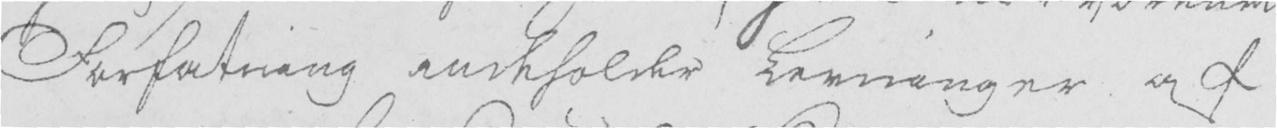Forfatning indeholder Levninger af
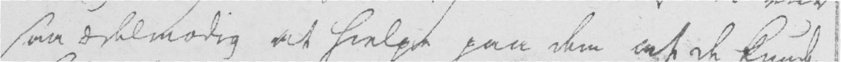saa ædelmodig at hielpe paa dem at de kunde
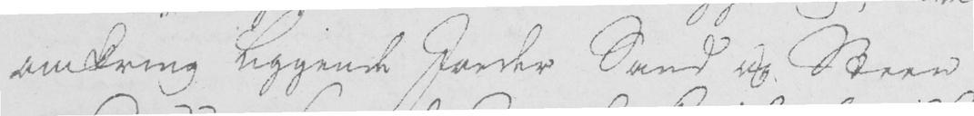omkring liggende havde Sand og Steen
